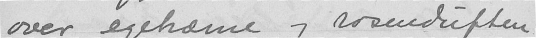over egetrærne og rosenduften
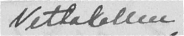Vettakollen
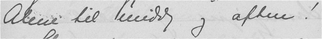Alene til middag og aften!
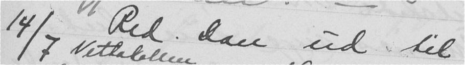14/7 Red Don ud til
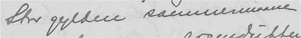Stor gylden sommermaane
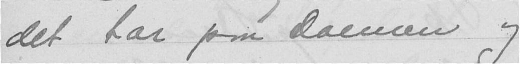det tar paa Donnen og
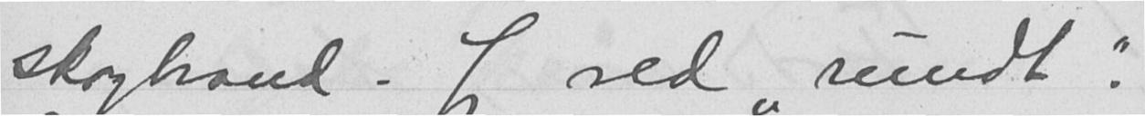skogbrand. Jeg red "rundt".
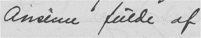Aviserne fulde af
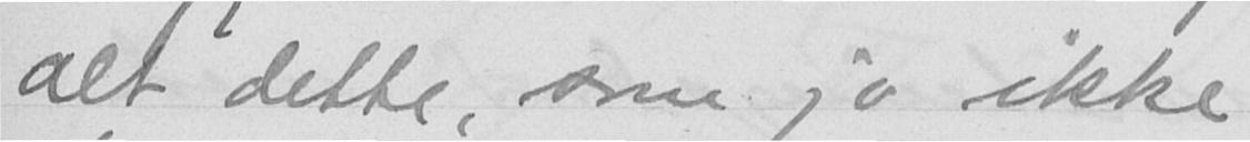alt dette, som jo ikke
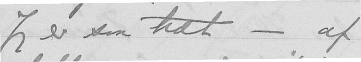Jeg er saa træt - af
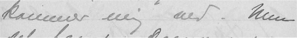kommer mig ved. Men
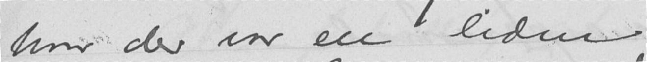hvor der var en liden
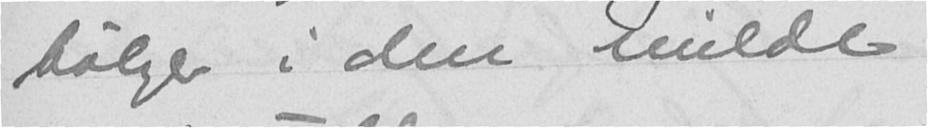bølger i den milde
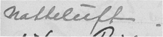natteluft.
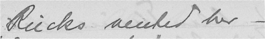Riecks vented her -
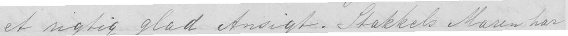et rigtig glad Ansigt. Stakkels Maren har
